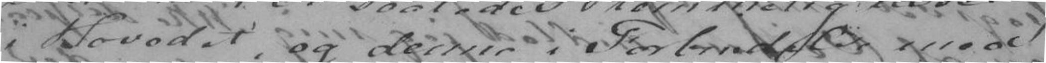i Hovedet, og denne i Forbindelse med
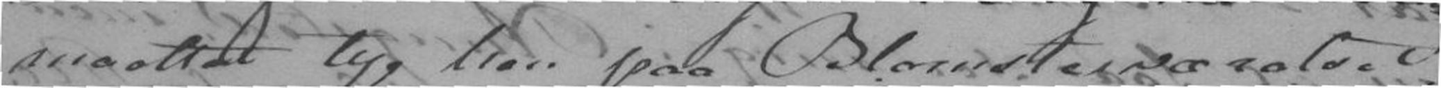maattet ty hen paa Blomsterværelset
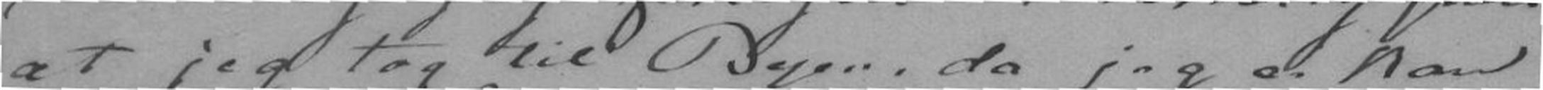at jeg tog til Byen, da jeg ei kan
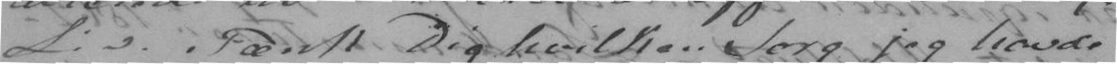Liv. Tænk Dig hvilken Sorg jeg havde
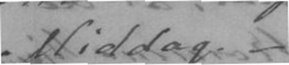Middag. -
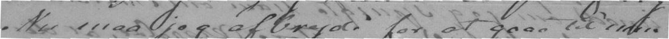Nu maa jeg afbryde for at gaae til min
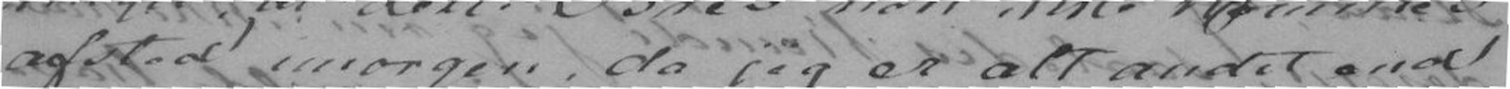afsted imorgen, da jeg er alt andet end
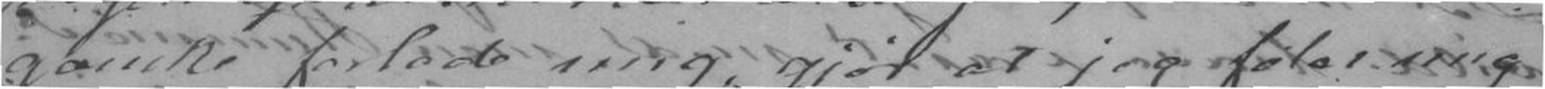ganske forlade mig, gjør at jeg føler mig
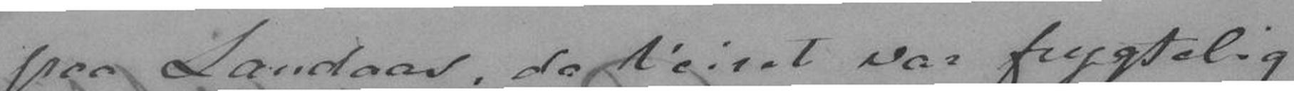paa Landaas, da Veiret var frygtelig
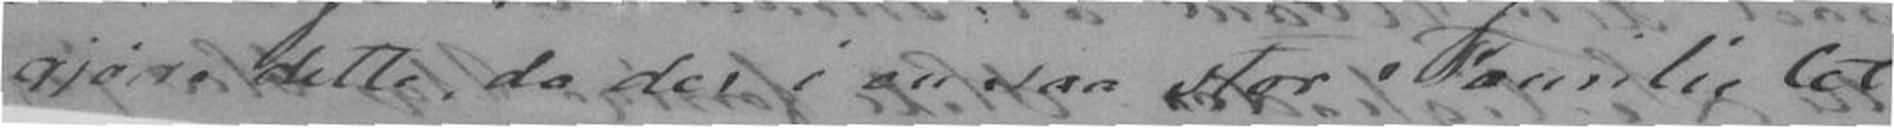gjøre dette, da der i en saa stor Familie let
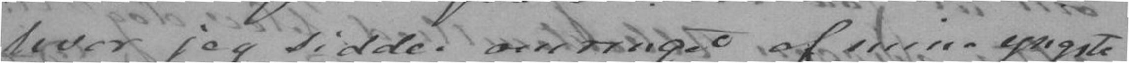hvor jeg sidder omringet af mine yngste
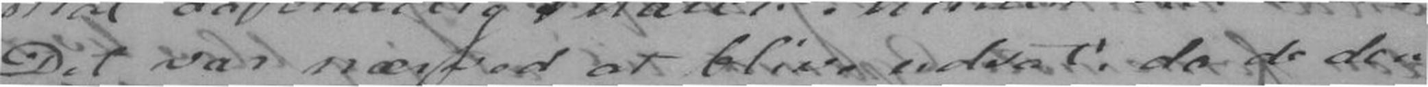Det var nærved at blive udsat, da de den
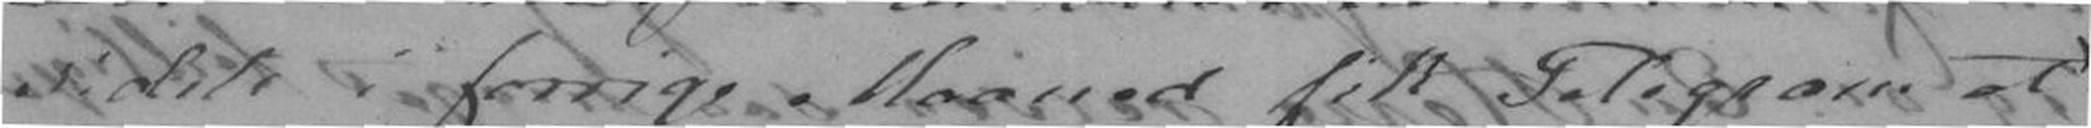sidste i forrige Maaned fik Telegram at
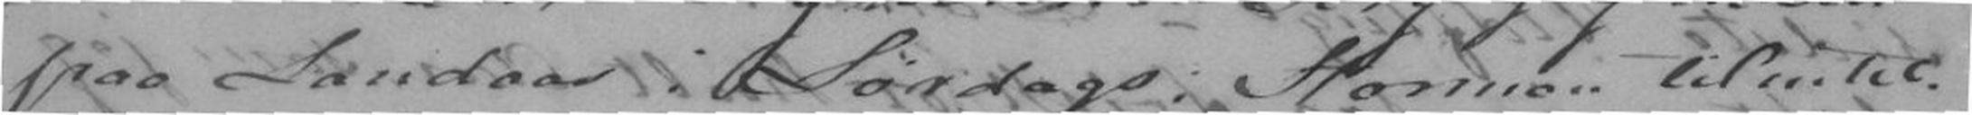paa Landaas i Lørdags; Stormen tilintet
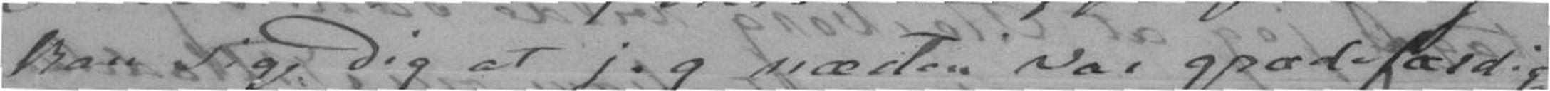kan sige at jeg næsten var grædefærdig.
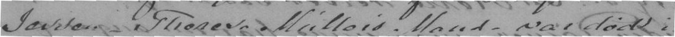Jesse-Therese Müllers Mand var død i
